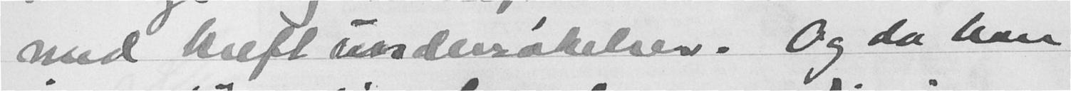med kreft undersøkelser. Og da han
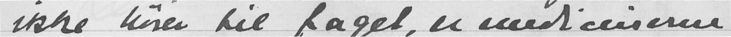ikke hører til faget, er medicenerne
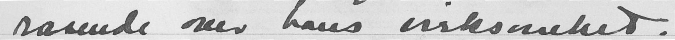rasende over hans virksomhet.
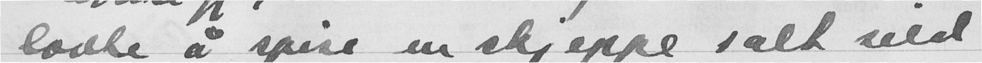lovte å spise en skjeppe salt sild
